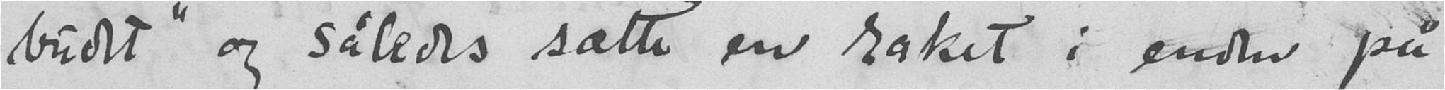budet" og således sætte en raket i enden på
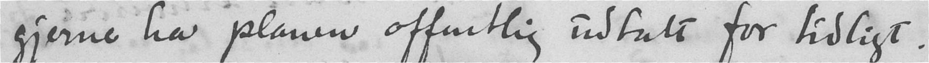gjerne ha planen offentlig udtalt for tidligt.
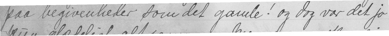paa begivenheder som det gamle! og dog var det jo
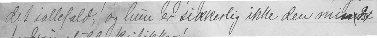det iallefald; og hun er sikkerlig ikke den mindste
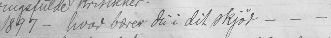1897 - hvad bærer du i dit skjød - - -
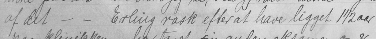af det - - Erling rask efter at have ligget 1 1/2 aar
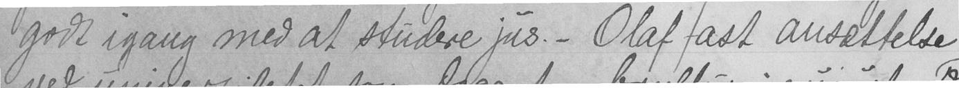godt igang med at studere jus. - Olaf fast ansættelse
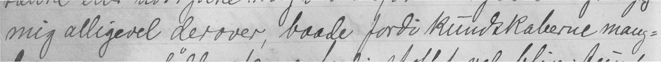mig alligevel derover, baade fordi kundskaberne mang-
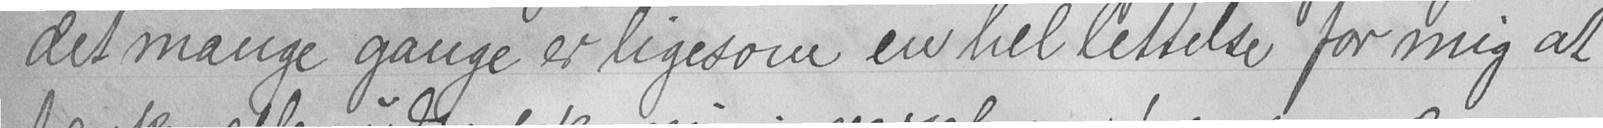det mange gange er ligesom en hel lettelse for mig at
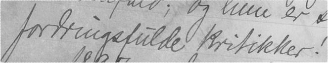fordringsfulde kritikker!
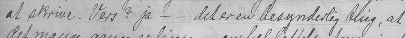at skrive. Vers? ja - - det er en besynderlig ting, at
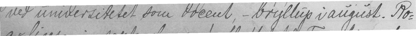ved universitetet som docent, - bryllup i august. Ro-
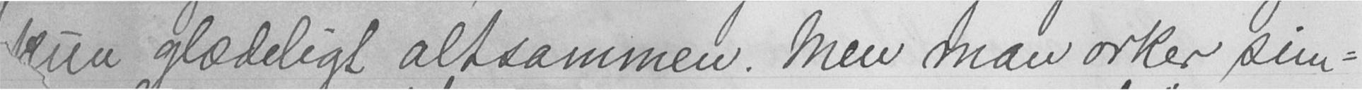kun glædeligt altsammen. Men man orker sim-
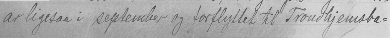ar ligesaa i september og forflyttet til Trondhjemsba-
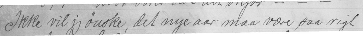Ikke vil jeg ønske, det nye aar maa være saa rigt
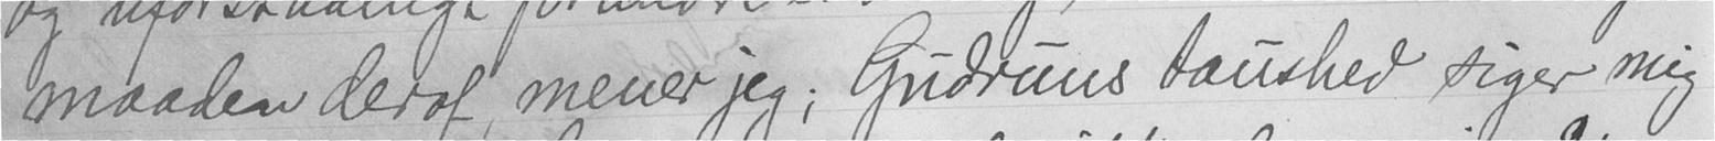maaden deraf, mener jeg; Gudruns taushed siger mig
